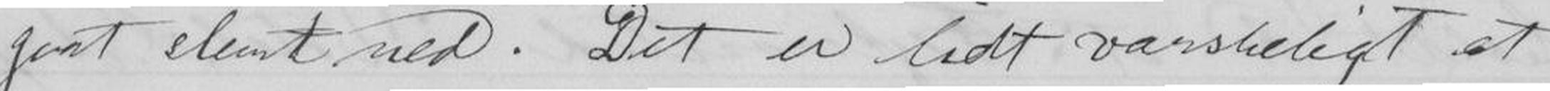gaat slemt ned. Det er lidt vanskeligt at
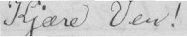Kjære Ven!
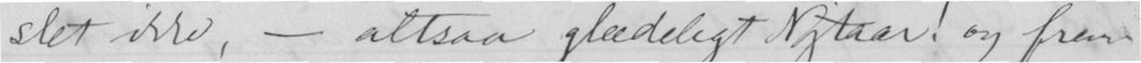slet ikke, - altsaa glædeligt Nytaar! og fram
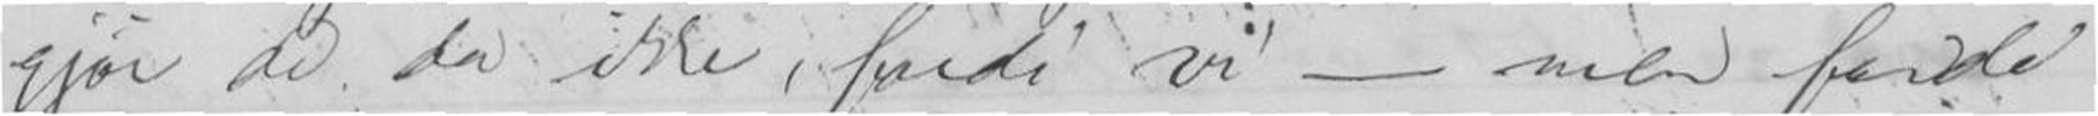gjør de da ikke, fordi vi - men fordi
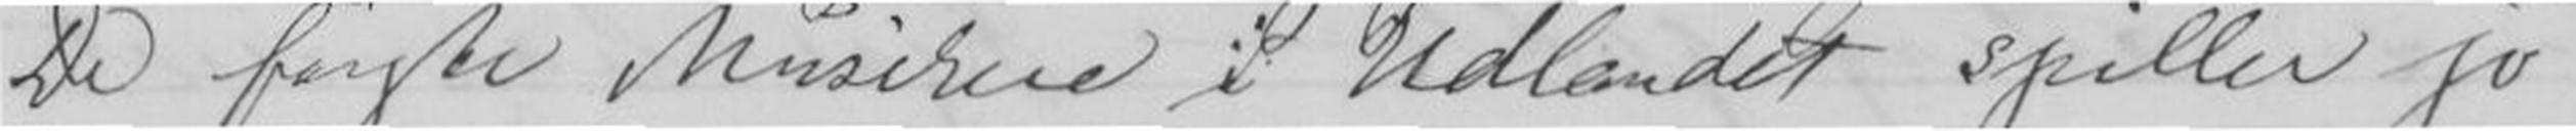De første Musikere i Udlandet spiller jo
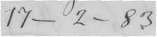17-2-83.
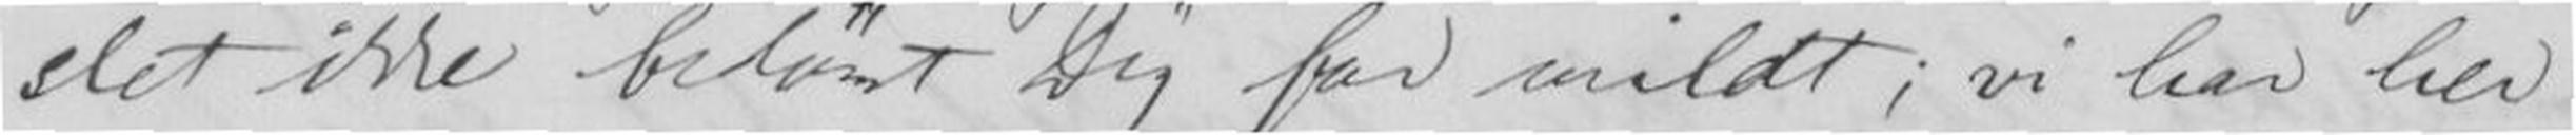slet ikke bedømt Dig for mildt; vi har her
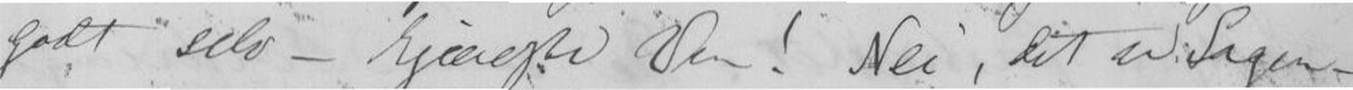godt selv - kjæreste Ven! Nei, det er Sagen
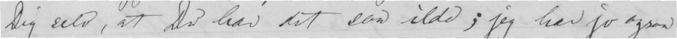Dig selv, at Du har det saa ilde; jeg har jo ogsaa
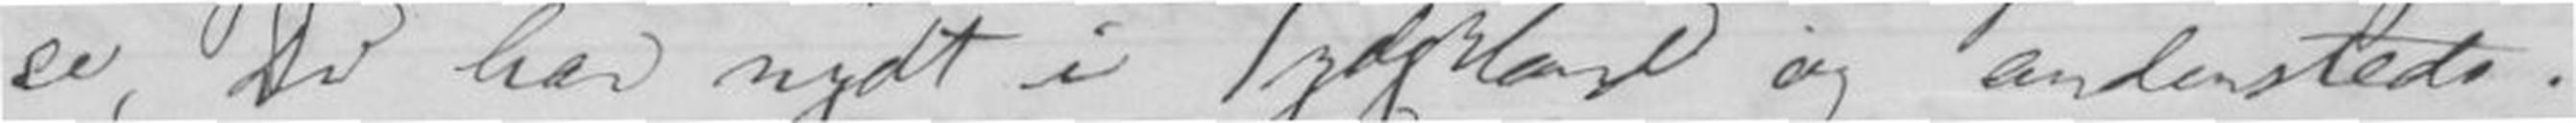se, Du har nydt i Tydskland og andensteds.
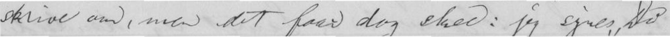skrive om, men det faar dog skee: jeg synes Du
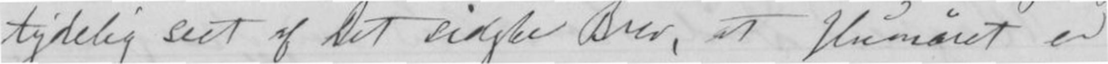tydeligt seet af Dit sidste Brev, at Humøret er
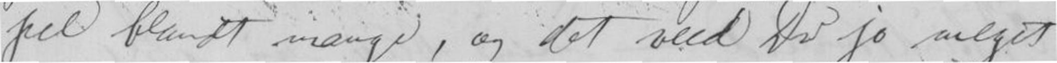pel blandt mange, og det veed Du jo meget
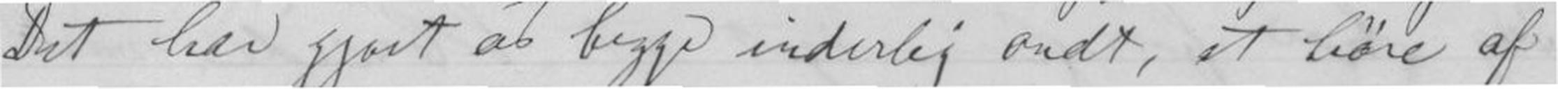Det har gjort os begge inderlig ondt, at høre af
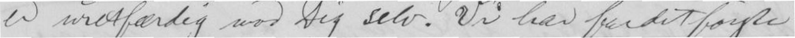er uretfærdig mod Dig selv. Vi har for det første
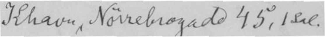Khavn, Nørrebrogade 45, 1 Sal.
